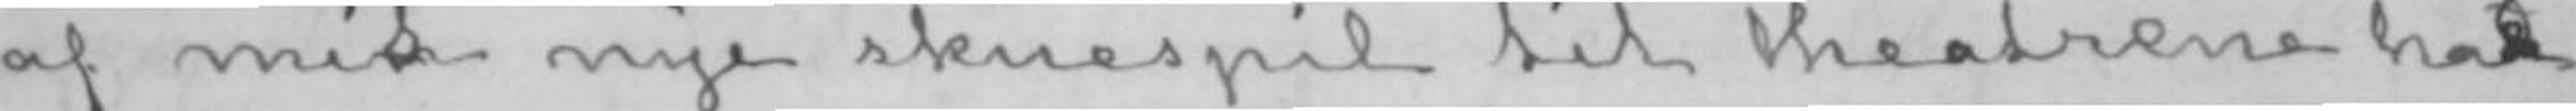af mit nye skuespil til theatrene har
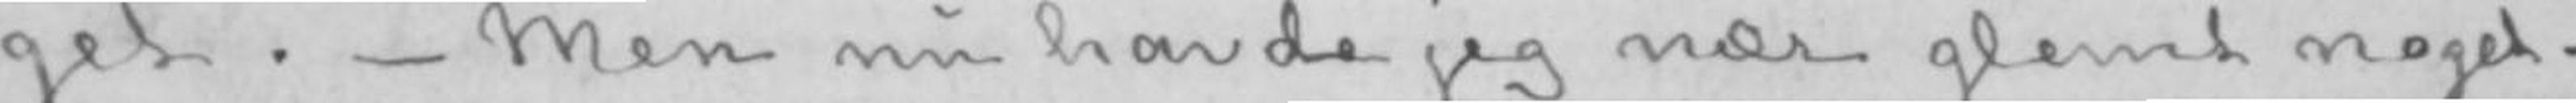get.- Men nu havde jeg nær glemt noget.
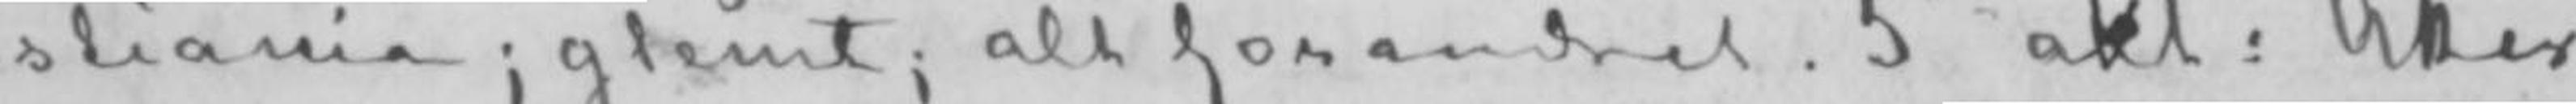stiania; glemt; alt forandret. 5te akt: Atter
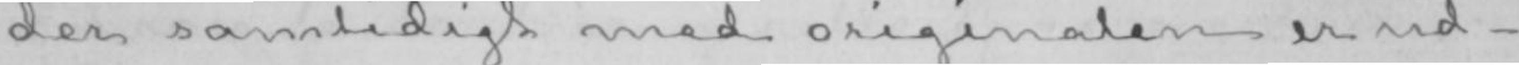der samtidigt med originalen er ud-
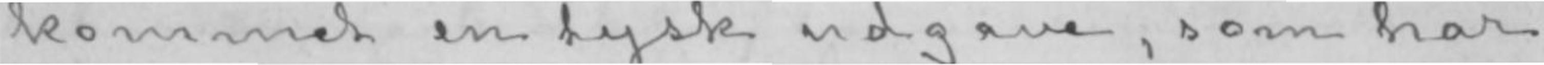kommet en tysk udgave, som har
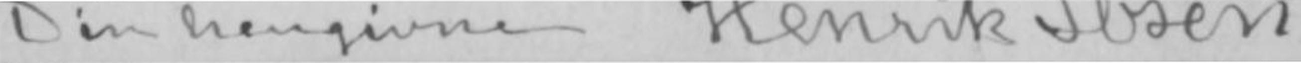Din hengivne Henrik Ibsen.
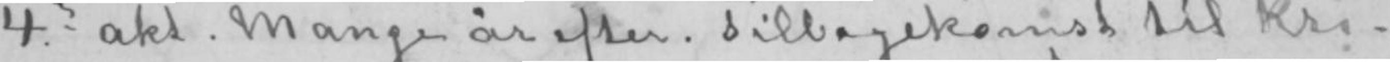4de akt. Mange år efter. Tilbagekomst til Kri-
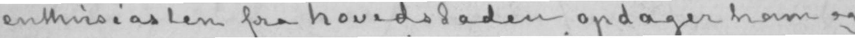enthusiasten fra hovedstaden opdager ham og
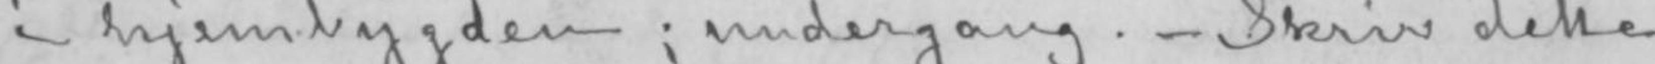i hjembygden; undergang.- Skriv dette
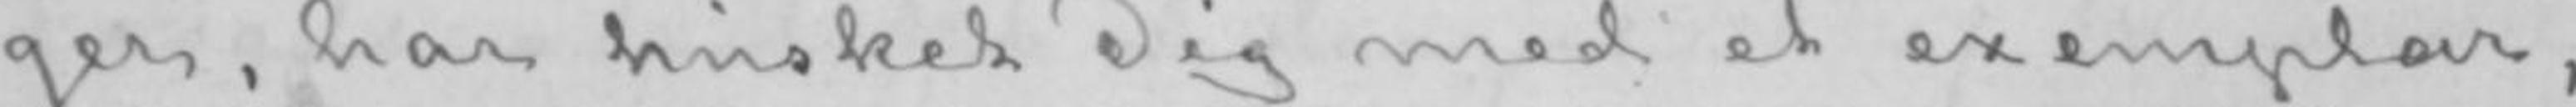ger, har husket Dig med et exemplar,
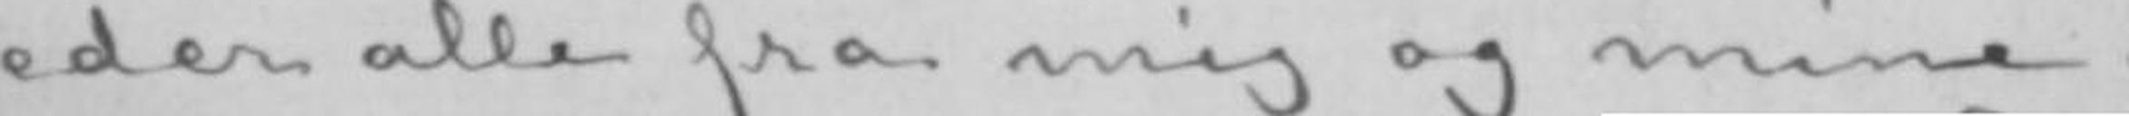eder alle fra mig og mine.
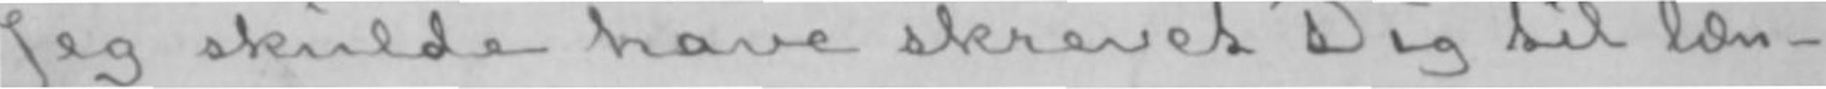Jeg skulde have skrevet Dig til læn-
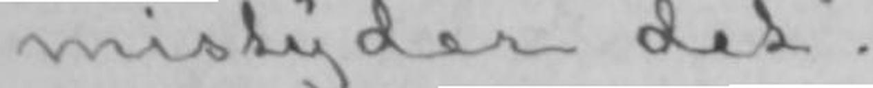mistyder det.
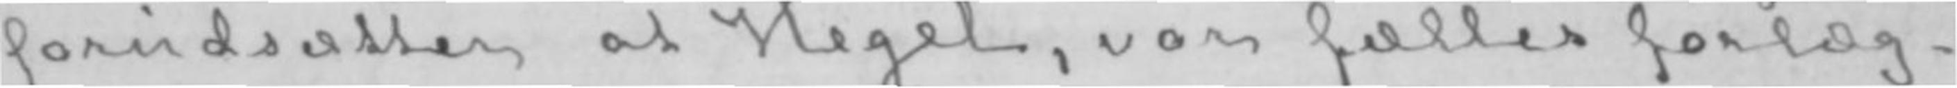forudsætter at Hegel, vor fælles forlæg-
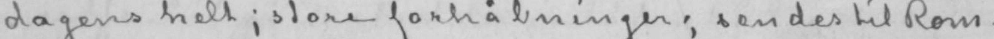dagens helt; store forhåbninger; sendes til Rom.
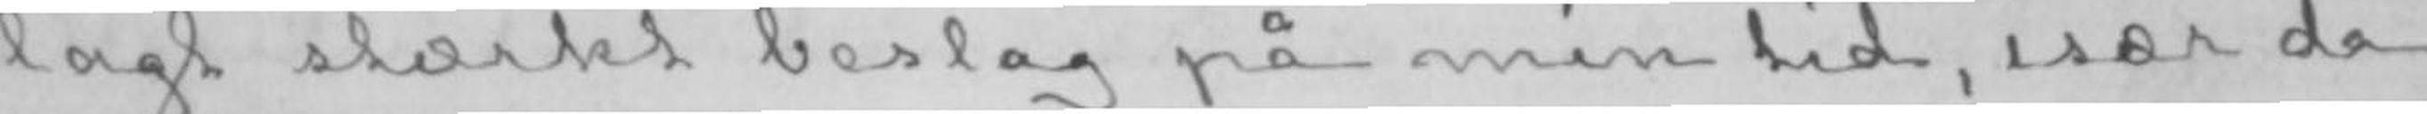lagt stærkt beslag på min tid, især da
